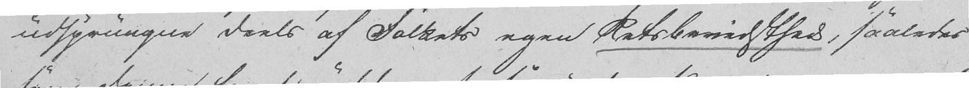udsprungne deels af Folkets egen Retsbevidsthed, saaledes
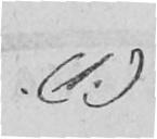(1.)
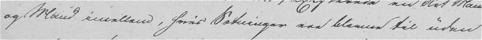og Mand imellem, hvis Sætninger ere blevne til uden
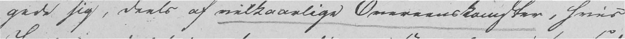gede sig, deels af vilkaarlige Overeenskomster, hvis
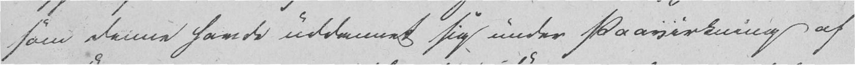som denne havde uddannet sig under Paavirkning af
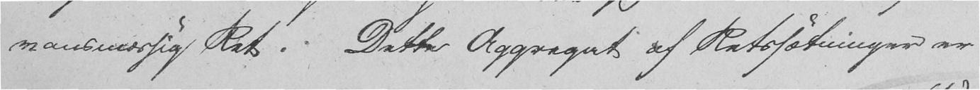vansmæssig Ret. Dette Aggregat af Retssætninger er
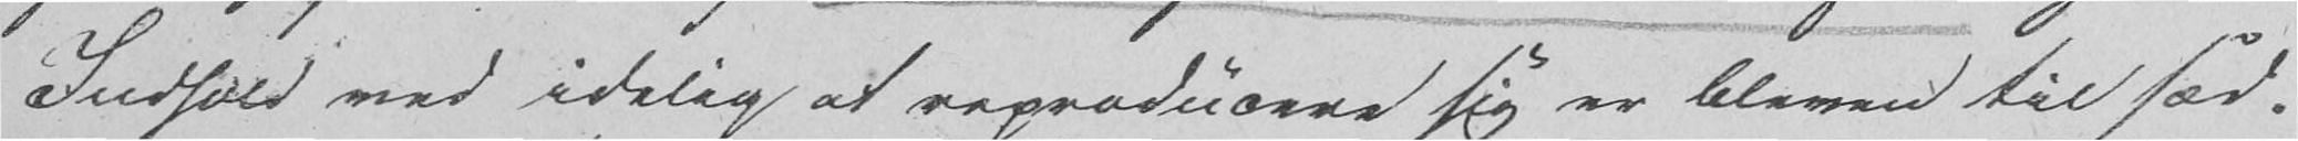Indhold ved idelig at reproducere sig er bleven til sæd-
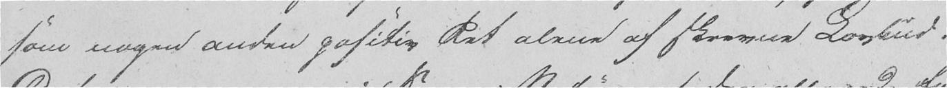som nogen anden positiv Ret alene af skrevne Lovbud.
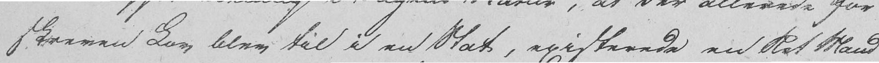skreven Lov blev til i en Stat, existerede en Ret Mand
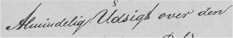Almindelig Udsigt over den
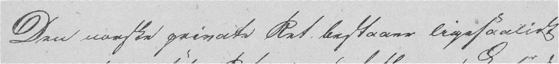Den norske private Ret bestaaer ligesaalidt
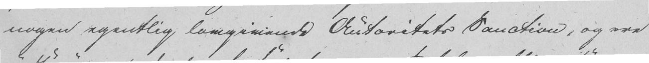nogen egentlig lovgivende Autoritets Sanction, og ere
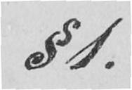§ 1.
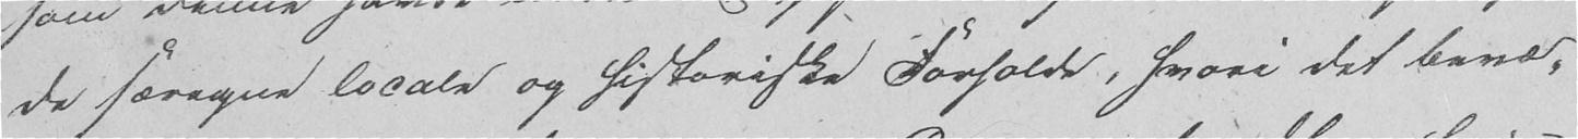de særegne locale og historiske Forholde, hvori det bevæ-
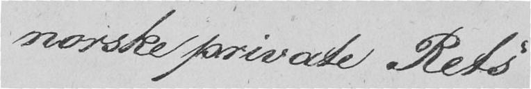norske private Rets
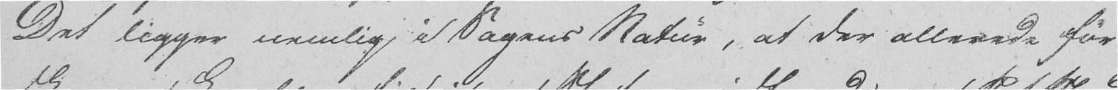Det ligger nemlig i Sagens Natur, at der allerede før
